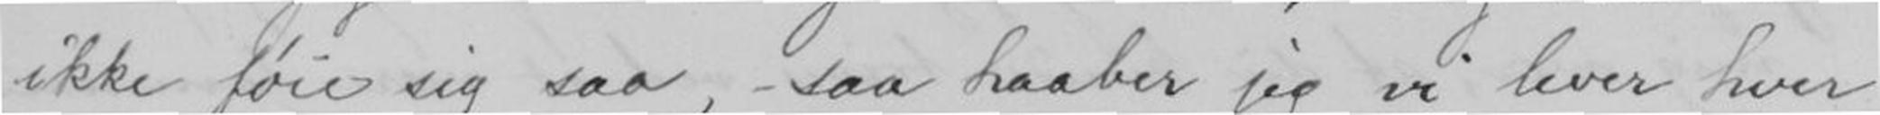ikke føre sig saa, - saa haaber jeg vi lever hver
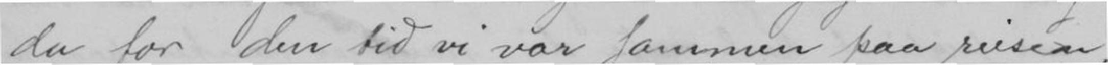da for den tid vi var sammen paa reise
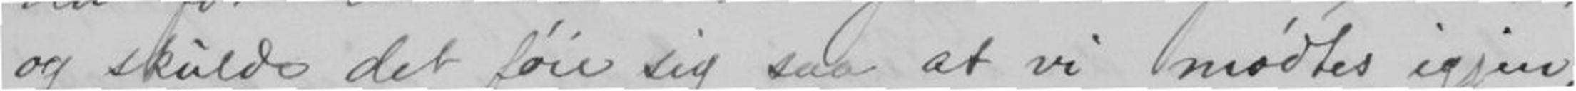og skulde det føre sig saa at vi mødtes igjen,
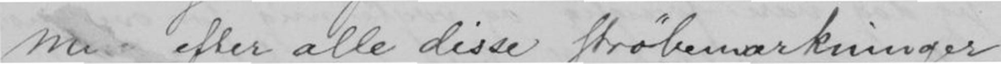Men efter alle disse strøbemærkninger
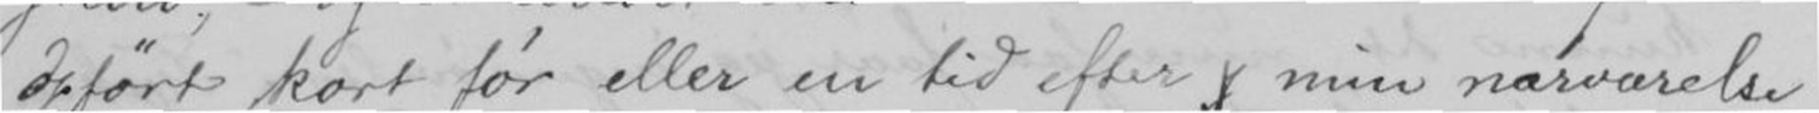opført kort før eller en tid efter min nærværelse
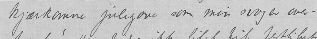kjærkomne julegave som min svoger over-
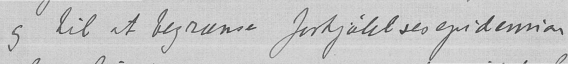og til at begrænse forkjølelsesepidemien
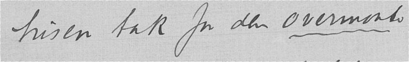tusen tak for den overmaate
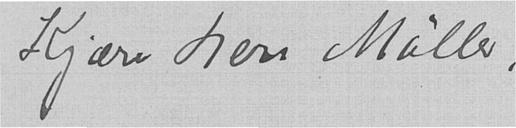Kjære herr Møller,
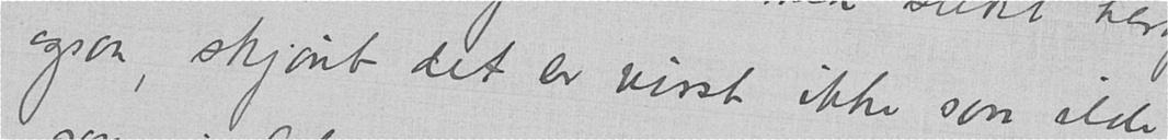ogsaa, skjønt det er visst ikke saa ilde
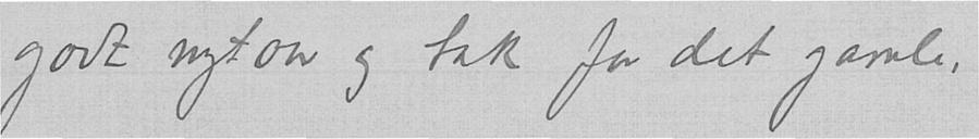godt nytaar og tak for det gamle,
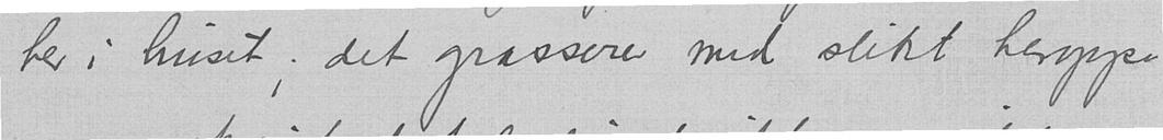her i huset; det grasserer med slekt heroppe
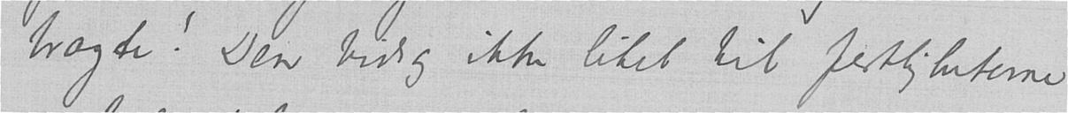bragte. Den bidrog ikke litet til festligheterne
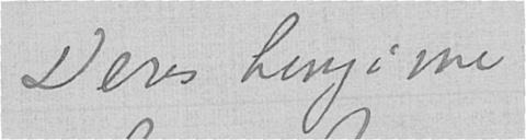Deres hengivne
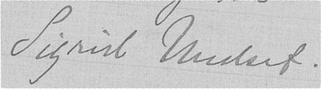Sigrid Undset.
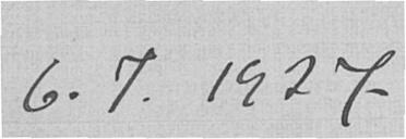6.7.1927.
